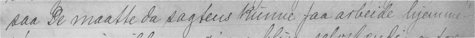saa De maatte da sagtens kunne faa arbeide hjemme - "
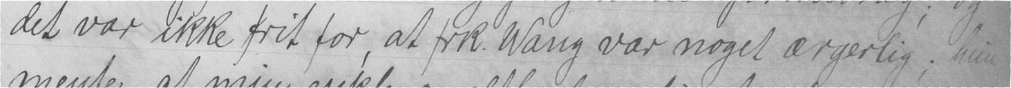det var ikke frit for, at frk. Wang var noget ærgerlig; hun
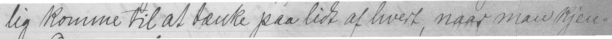lig komme til at tænke paa lidt af hvert, naar man kjen-
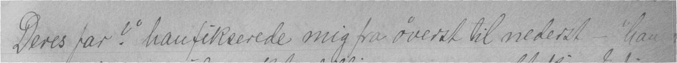Deres far?" han fikserede mig fra øverst til nederst - "han
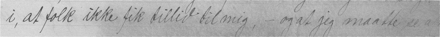i, at folk ikke fik tillid til mig; - og at jeg maatte se at
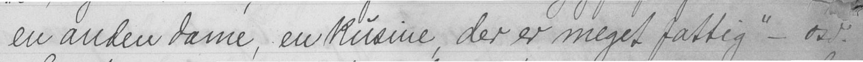en anden dame, en kusine, der er meget fattig" - osv.
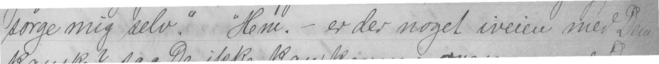sørge mig selv."  "Hem. - er der noget iveien med Dem
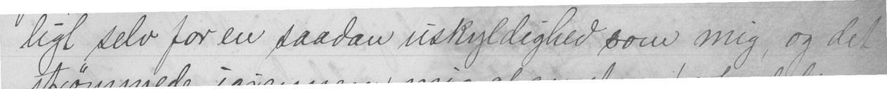ligt selv for en saadan uskyldighed som mig, og det
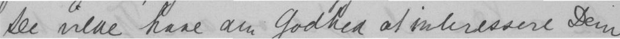De vilde have den Godhed at interessere Dem
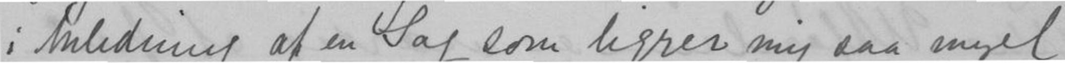i Anledning af en Sag som ligger mig saa meget
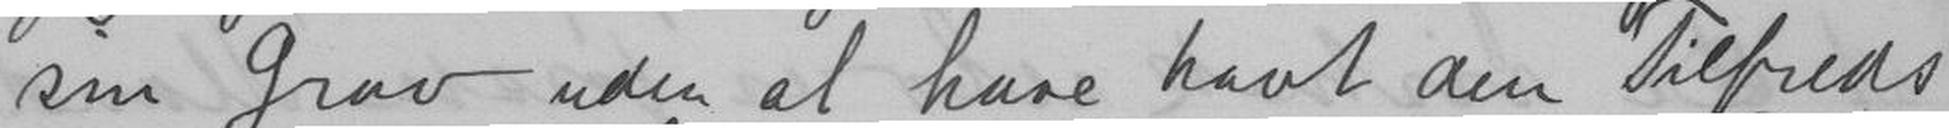sin Grav uden at have havt den Tilfreds
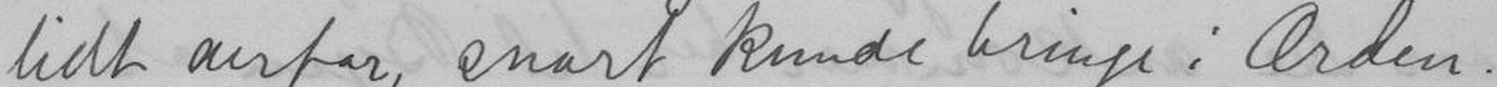lidt derfor, snart kunde bringe i Orden.
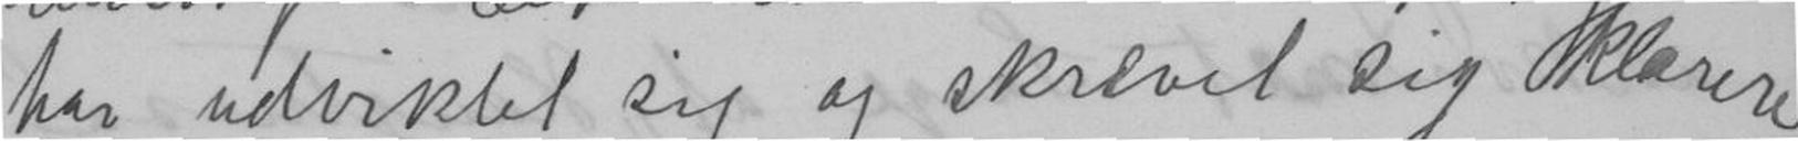har udviklet sig og skrevet sig klarere
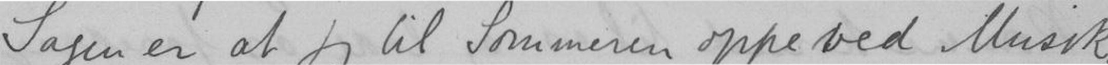Sagen er at jeg til Sommeren oppeved Musik-
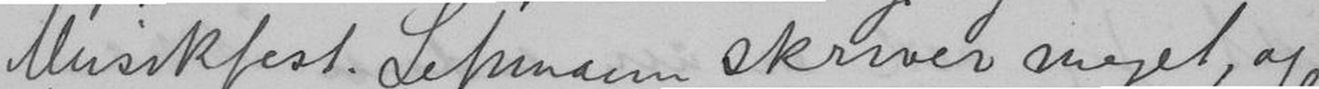Musikfest. Lehmann skriver meget, og
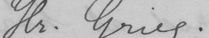Hr. Grieg.
